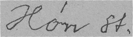Høn St.
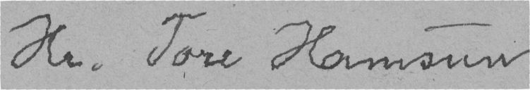Hr. Tore Hamsun
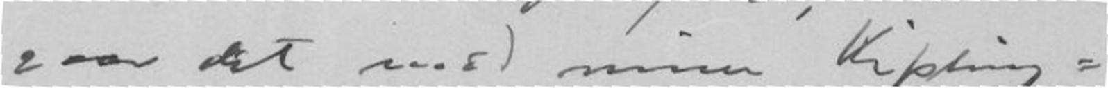gaar det med min Kiplin-
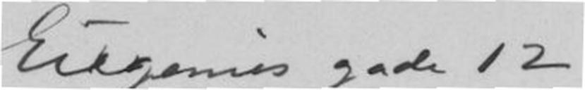Eugenis gade 12
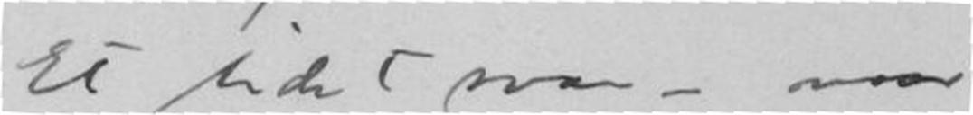Et lide svar - naar
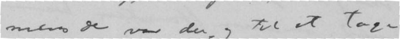mens de var der til at tage
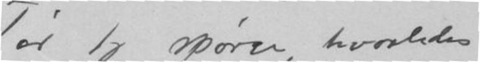Tør jeg spørre, hvorledes
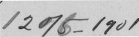12/6 1901
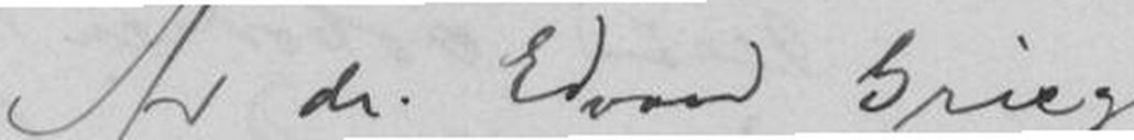Hr dr. Edvard Grieg
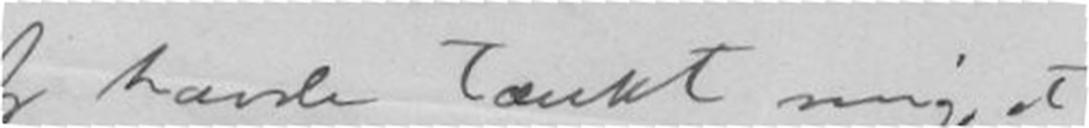Jeg havde tænkt mig, at
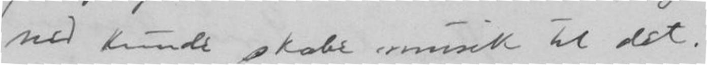hed kunde skabe musik til det.
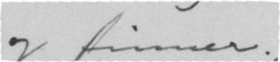og finner.
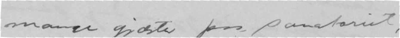mange gjæster paa Sanatoriet,
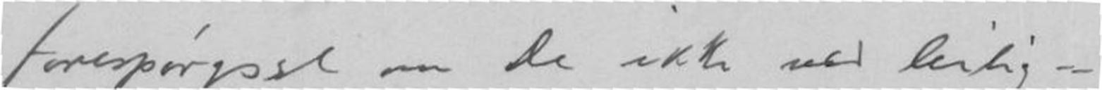forespørgsel om De ikke ved leilig-
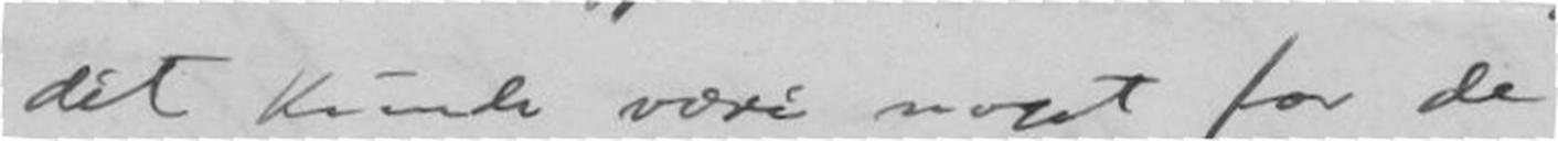det kunde være noget for de
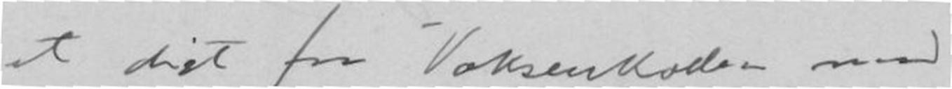et digt fra Voksenkollen med
